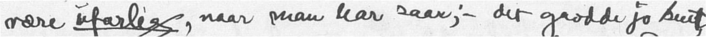være ufarlig, naar man har saar; - det grodde jo sent
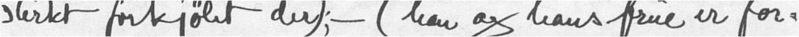sterkt forkjølet der); - (han og hans frue er for-
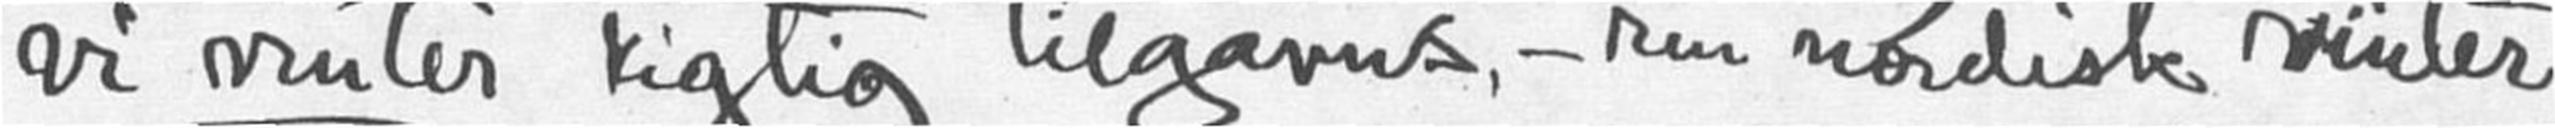vi vinter rigtig tilgavns, - ren nordisk vinter
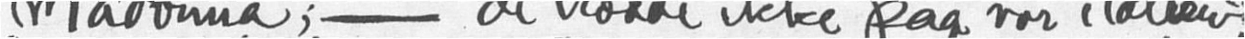og "Madonna"; - de trodde ikke paa vor italien-
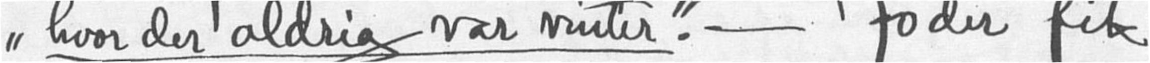"hvor der aldrig var vinter". - Jo der fik
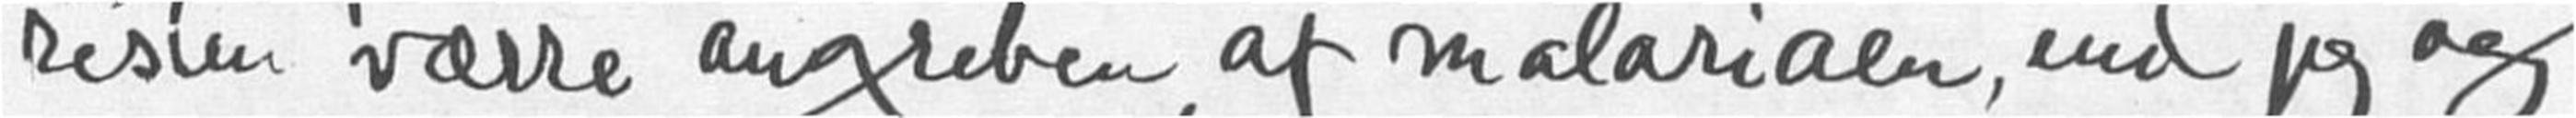resten værre angreben af malariaen, end jeg
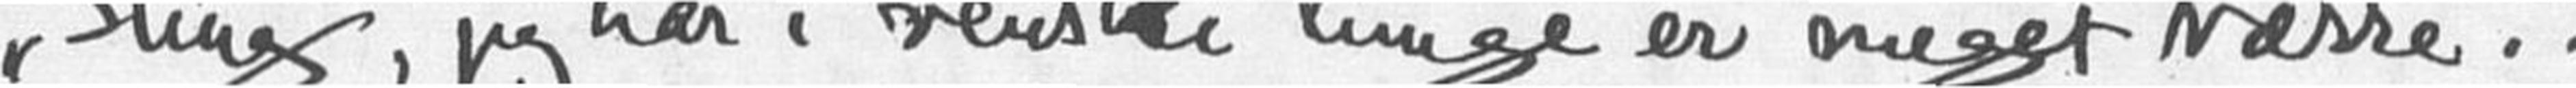"sting" jeg har i venstre lunge er meget værre. -
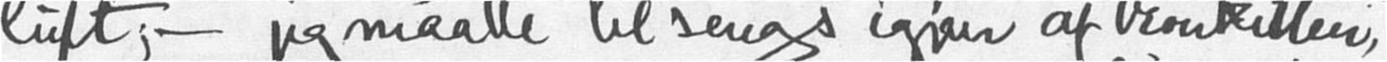luft, - jeg maatte tilsengs igjen af bronkitten
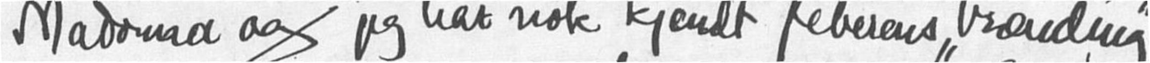Madonna og jeg har nok kjendt feberens brænding
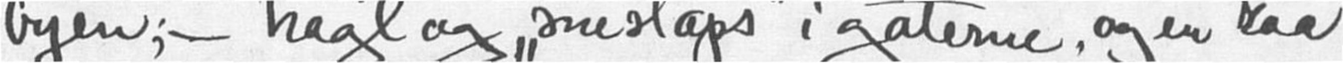byen; - hagl og "sneslaps" i gaterne, og en raa
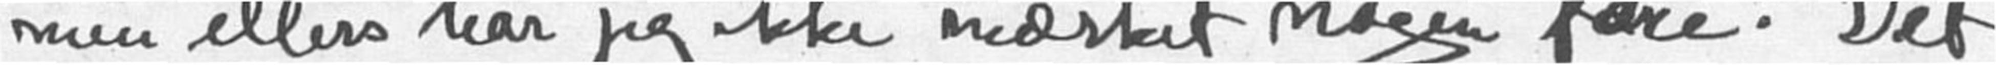men ellers har jeg ikke mærket nogen fare. Det
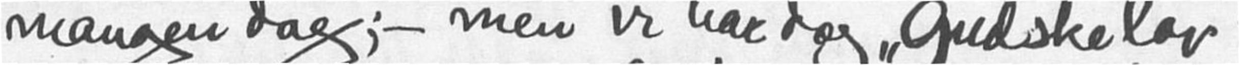mangen dag; - men vi har dog "gudskelov"
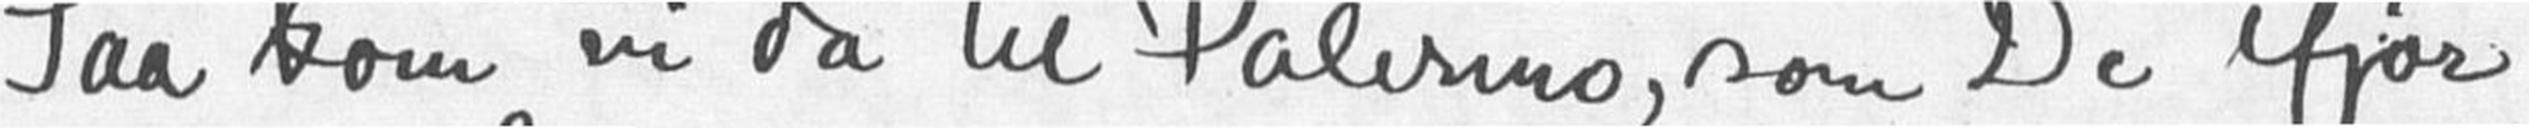Saa kom vi da til Palermo, som De ifjor
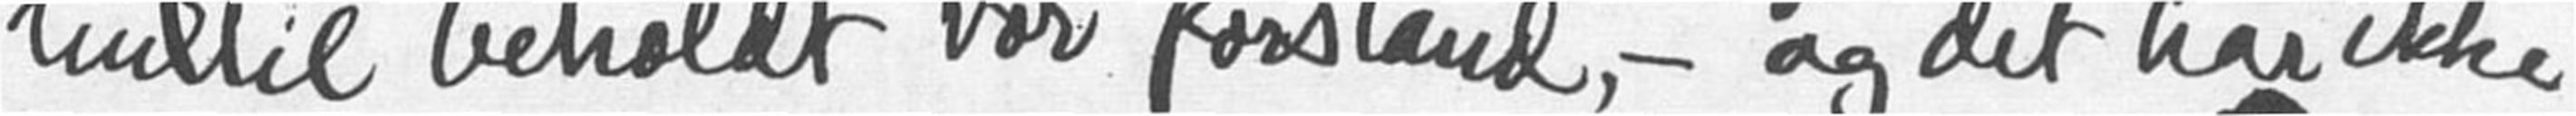hidtil beholdt vor forstand, og det har ikke
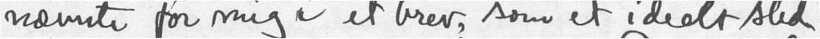nævnte for mig i et brev, som et ideelt sted
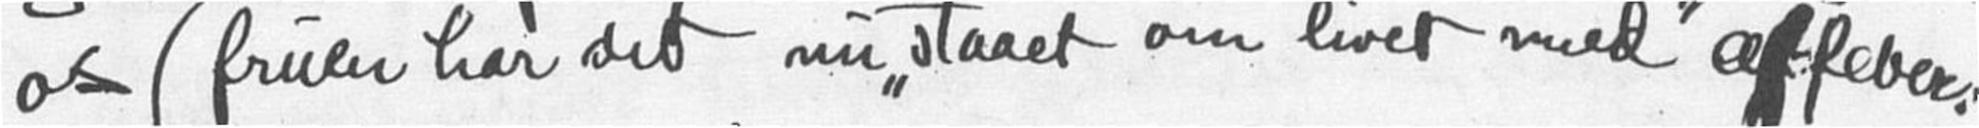os (fruen har det nu "staaet om livet med" af feber).
